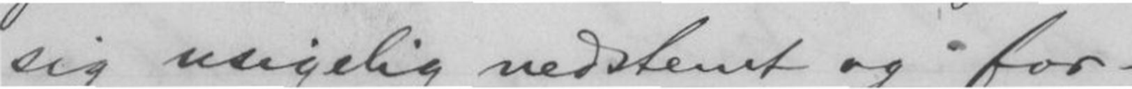sig usigelig nedstemt og for-
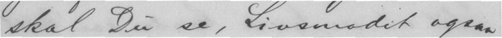skal Du se, Livsmodet ogsaa
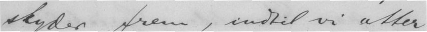skyder frem, indtil vi atter
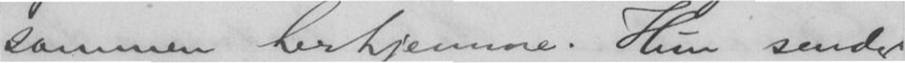sammen herhjemme. Hun sender
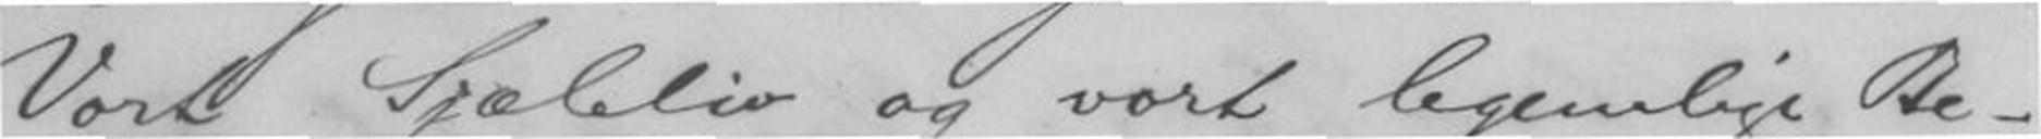Vort Sjæleliv og vort legemlige Be-
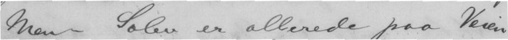Men Solen er allerede paa Veien
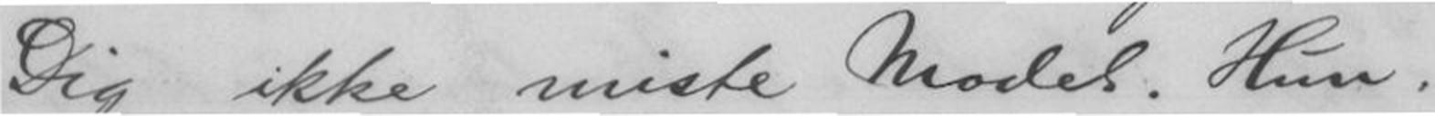Dig ikkr miste Modet. Hun
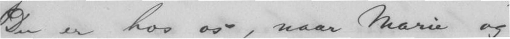Du er hos os, naar Marie og
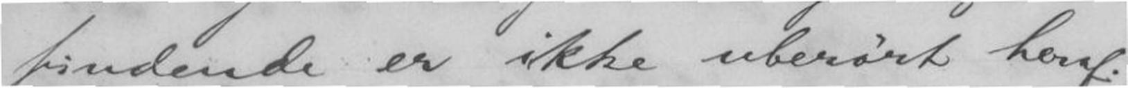findende er ikke uberørt heraf.
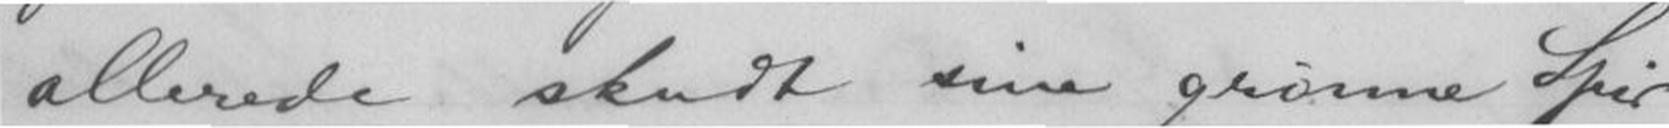allerede skudt sine grønne Spir
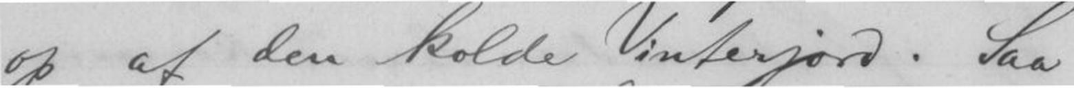op af den kolde Vinterjord. Saa
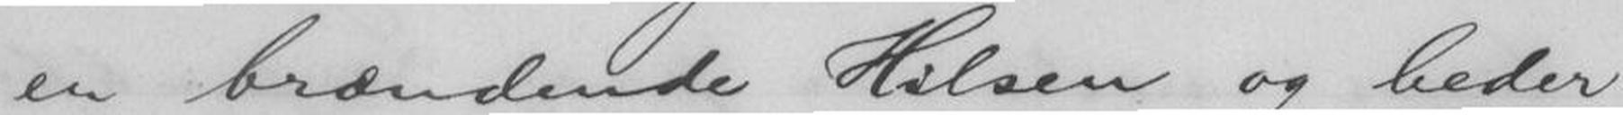en brændende Hilsen og beder
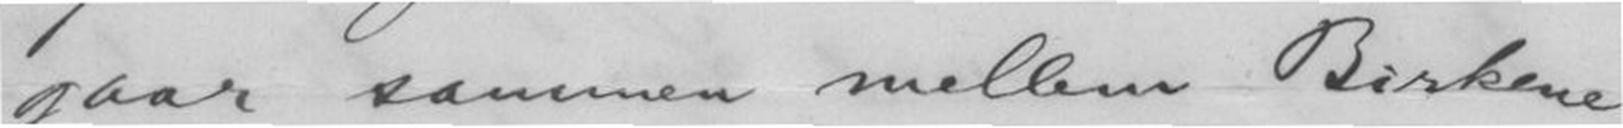gaar sammen mellem Birkene
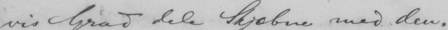vis Grad dele Skjæbne med den.
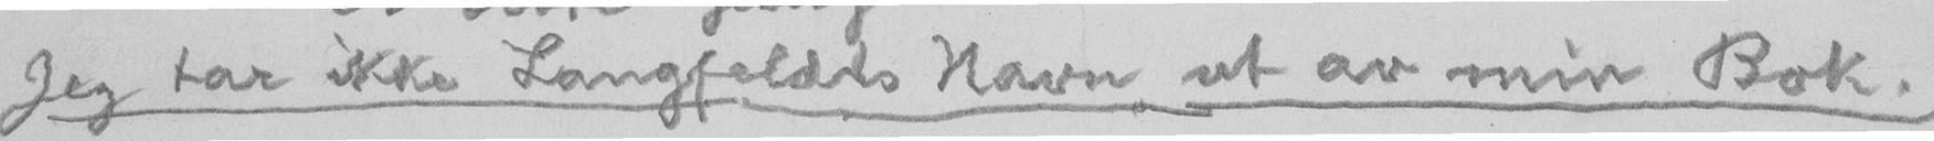Jeg tar ikke Langfeldts Navn ut av min Bok.
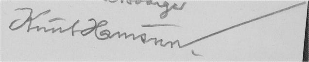Knut Hamsun.
